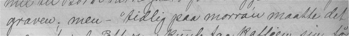graven; men - "tidlig paa morra'n maatte det
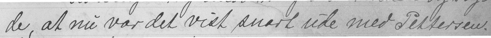de, at nu var det vist snart ude med Pettersen.
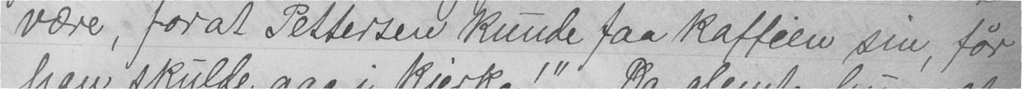være, for at Pettersen kunde faa kaffien sin, før
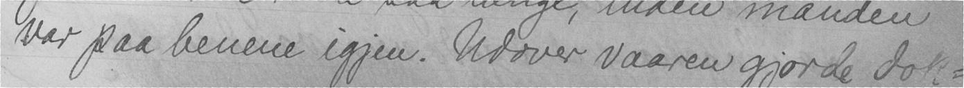var paa benene igjen. Udover vaaren gjorde dok-
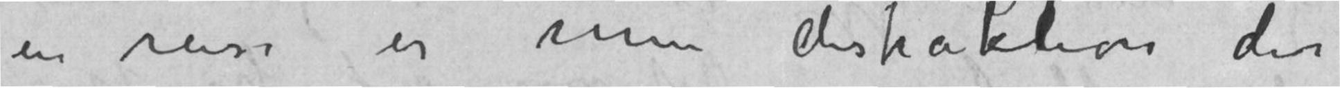en reise er min distraktion der
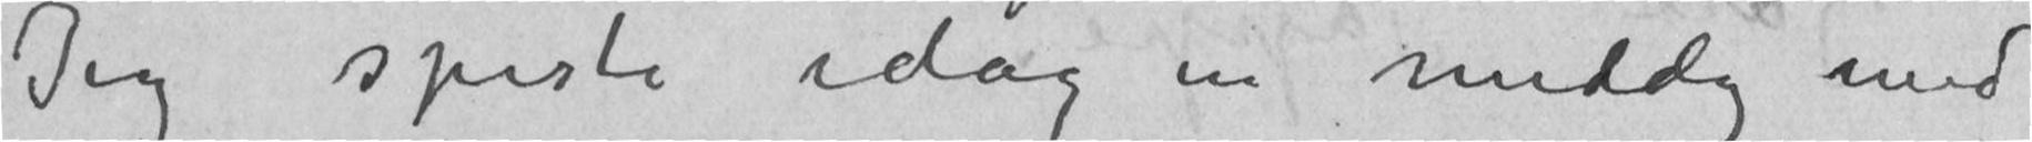Jeg spiste idag en middag med
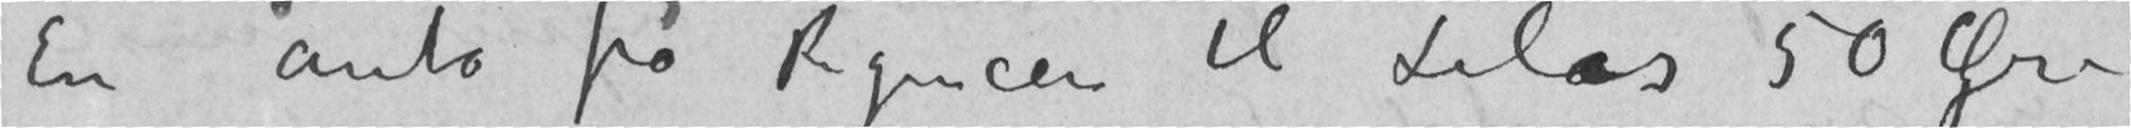En auto fra Regence til Lilas 50 Øre
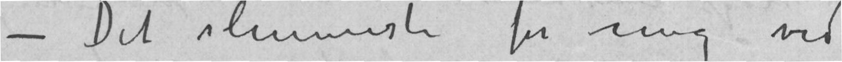– Det slemmeste for mig ved
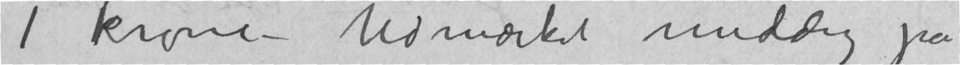1 krone – Udmærket middag på
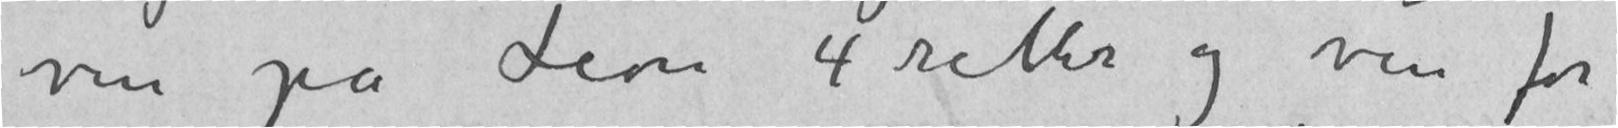vin på Leon 4 retter og vin for
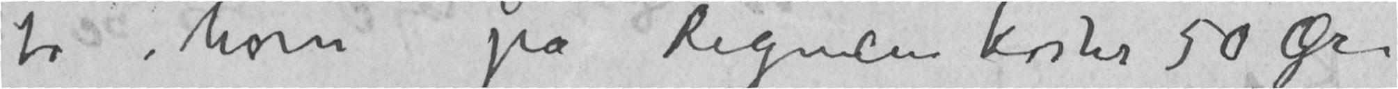to horn på Regence koster 50 øre
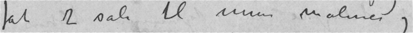fåt 2 sale til mine malerier og
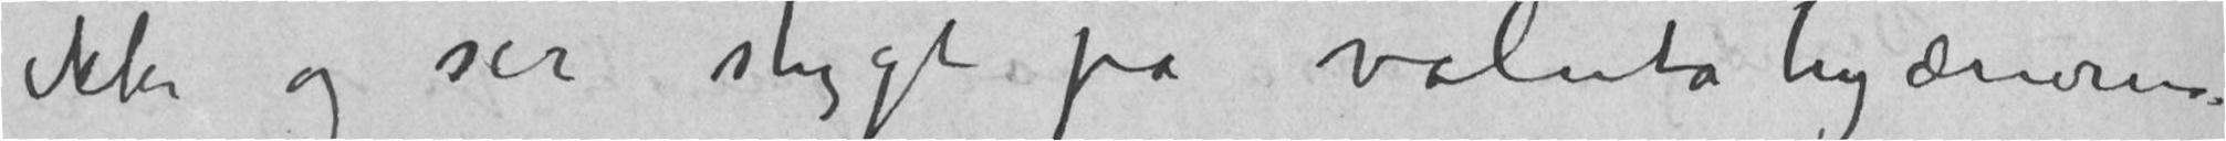ikke og ser stygt på valutahyænerne
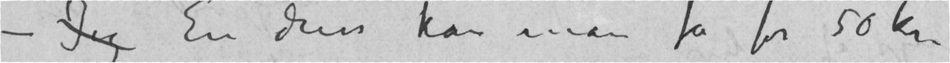– Jeg En dress kan man få for 50 kr
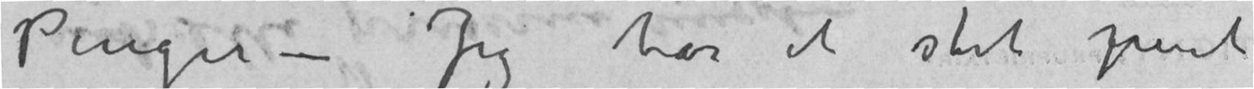Penger – Jeg har et stort pent
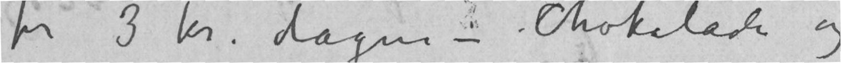for 3 kr. dagen – Chokolade og
